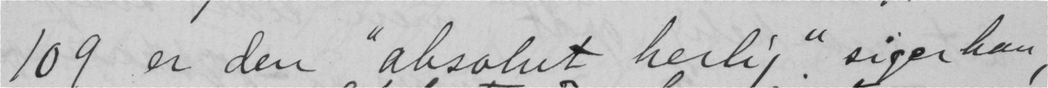109 er den "absolut herlig" siger han,
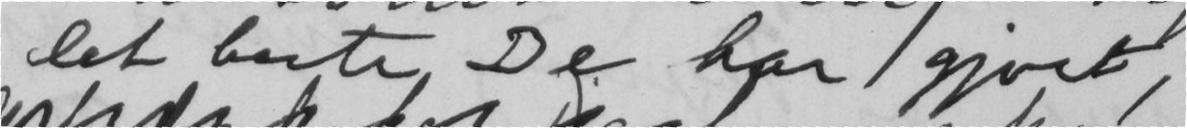det beste De har gjort,
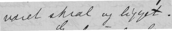været skral og ligget.
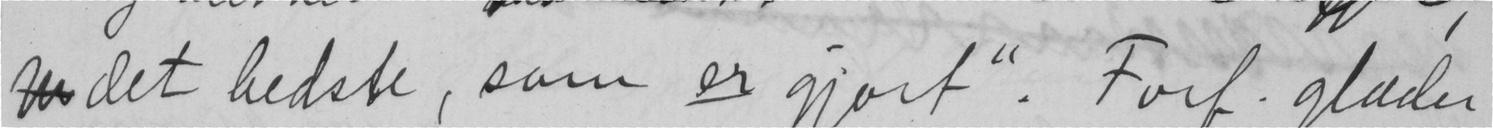det bedste, som er gjort". Forf. glæder
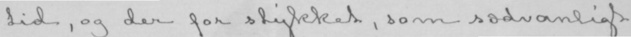tid, og der for stykket, som sædvanligt,
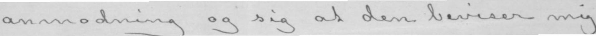anmodning og sig at den beviser mig
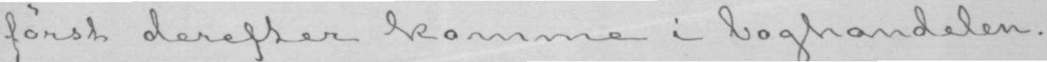først derefter komme i boghandelen.
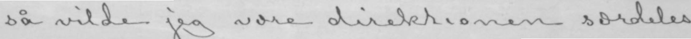så vilde jeg være direktionen særdeles
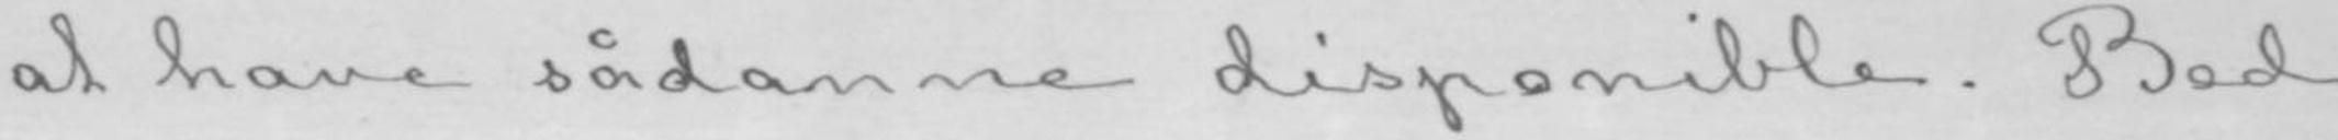at have sådanne disponible. Bed
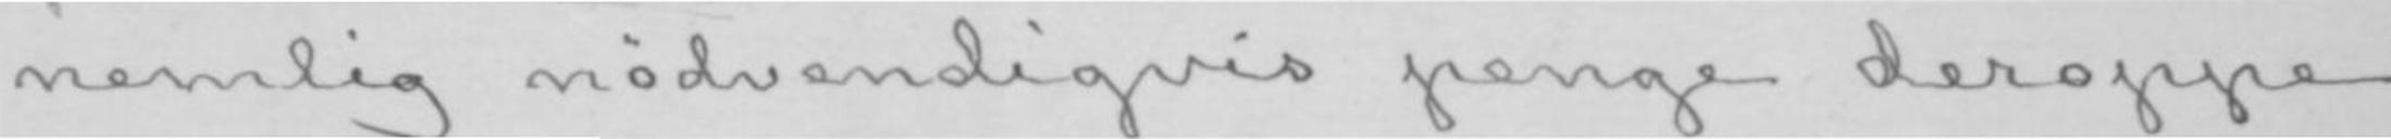nemlig nødvendigvis penge deroppe
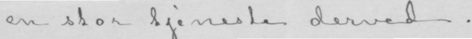en stor tjeneste derved.
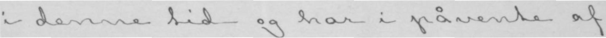i denne tid og har i påvente af
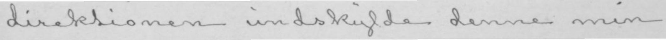direktionen undskylde denne min
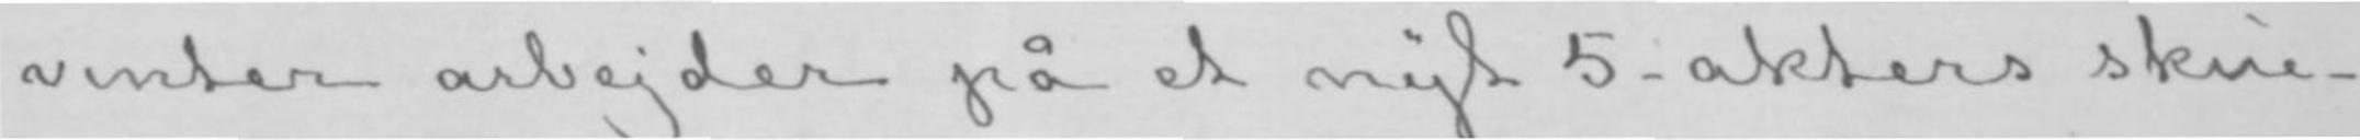vinter arbejder på et nyt 5-akters skue-
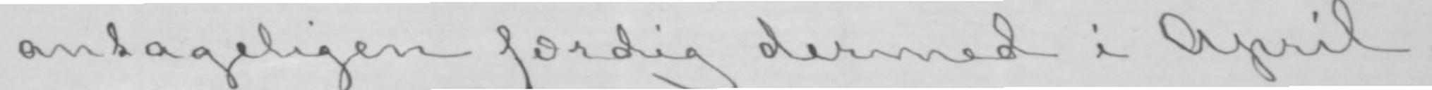antageligen færdig dermed i April.
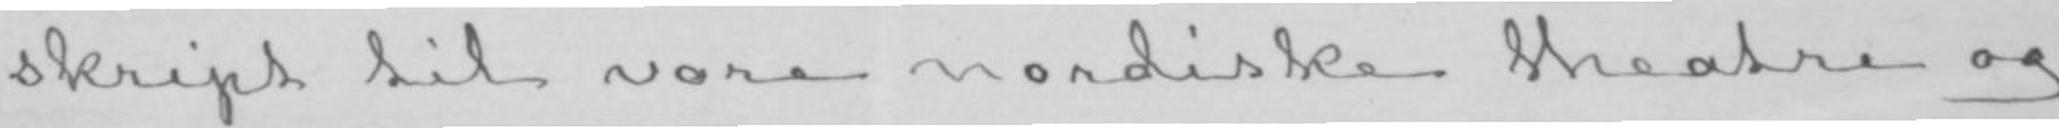skript til vore nordiske theatre og
